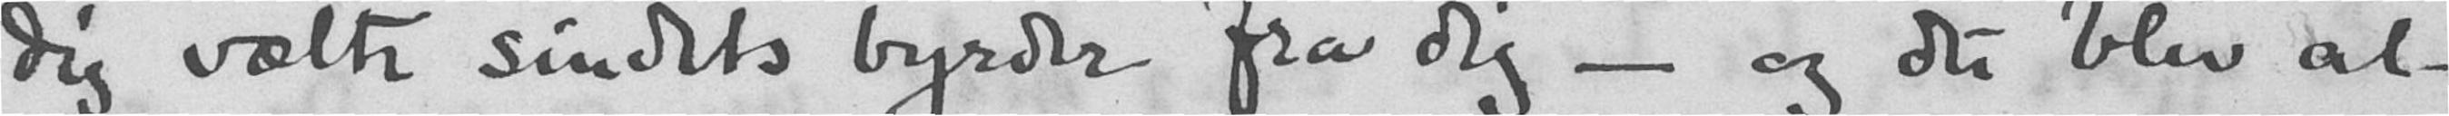dig vælte sindets byrder fra dig - og det blev al-
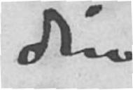din
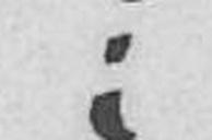i
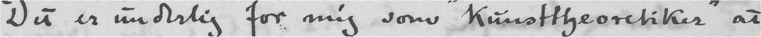Det er underlig for mig som "kunsttheoretiker" at
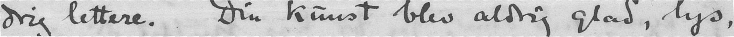drig lettere. Din kunst blev aldrig glad, lys,
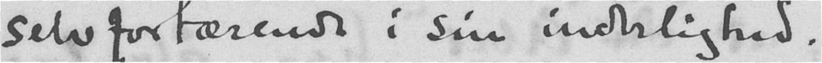selvfortærende i sin inderlighed.
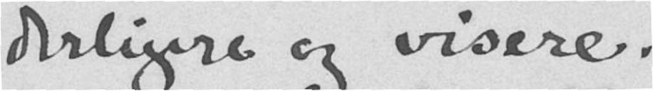derligere og visere.
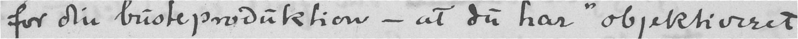for din busteproduktion - at du har "objektiveret"
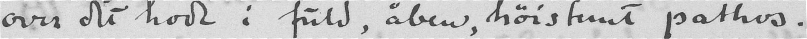over dit hode i fuld, åben, høistemt pathos.
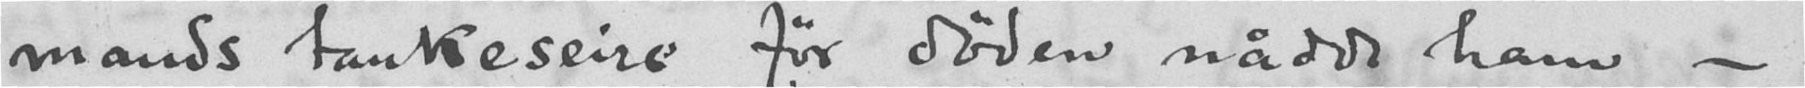mands tankeseire før døden nådde ham
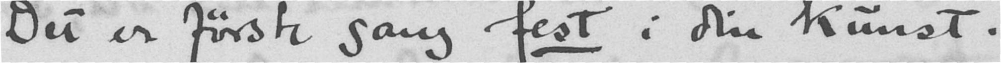Det er første gang fest i din kunst.
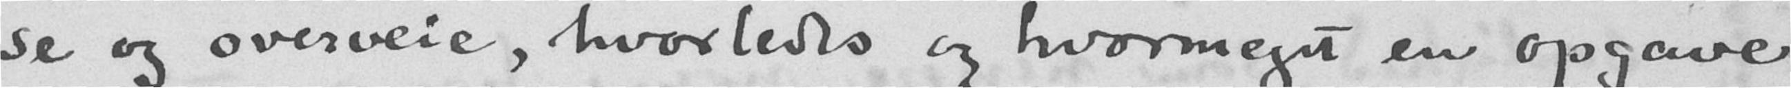se og overveie, hvorledes og hvormeget en opgave
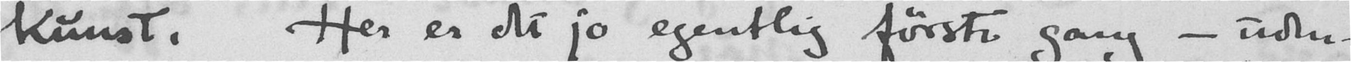kunst. Her er det jo egentlig første gang - uden-
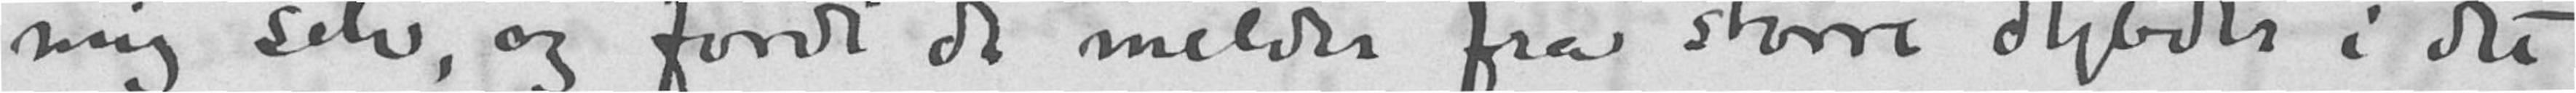mig selv, og fordi de melder fra større dybder i dit
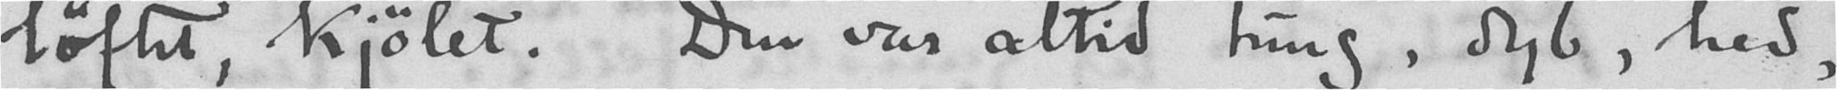løftet, kjølet. Den var altid tung, dyb, hed,
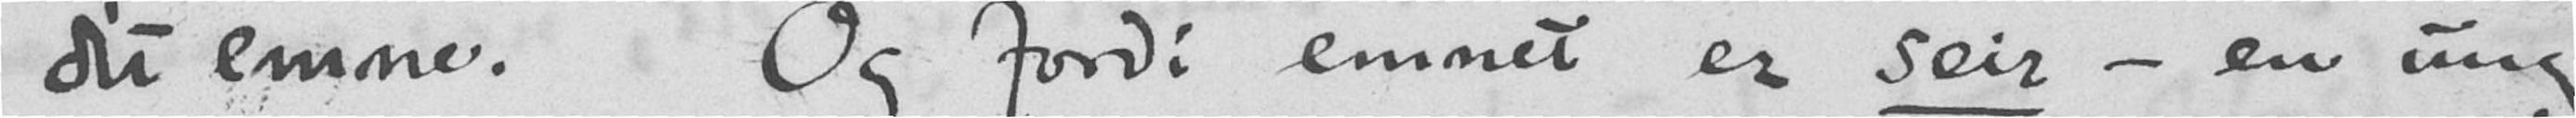dit emne. Og fordi emnet er seir - en ung
